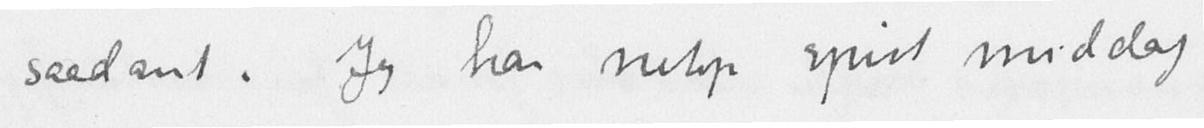saadant. Jeg har netop spist middag
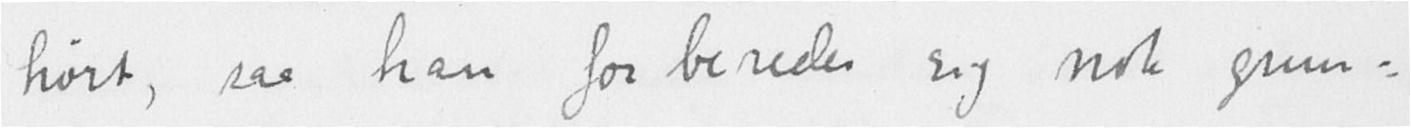hørt, saa han forbereder sig nok grun-
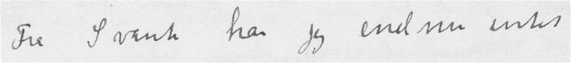Fra Svante har jeg endnu intet
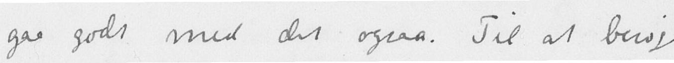gaa godt med det ogsaa. Til at besøge
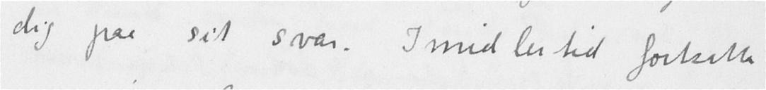dig paa sit svar. Imidlertid fortsætter
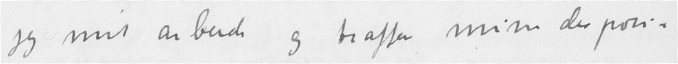jeg mit arbeide og træffer mine disposi-
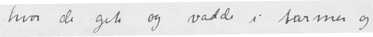hvor de gik og vadde i tarmer og
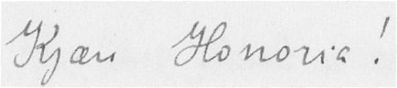Kjære Honoria!
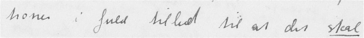tioner i fuld tillid til at det skal
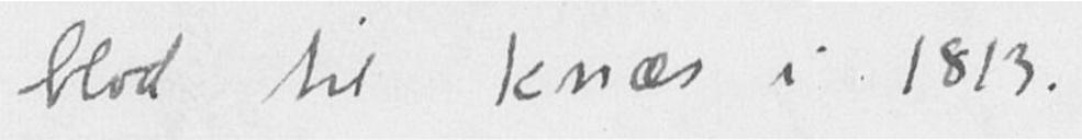blod til knæs i 1813.
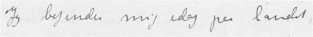Jeg befinder mig idag paa landet,
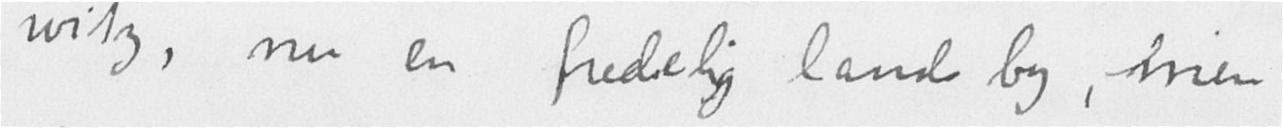witz, nu en fredelig landsby, men
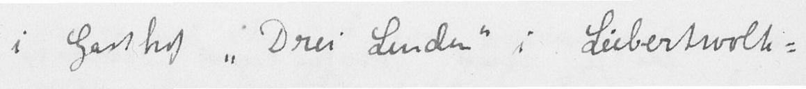i Gasthof "Drei Linden" i Liebertwolk-
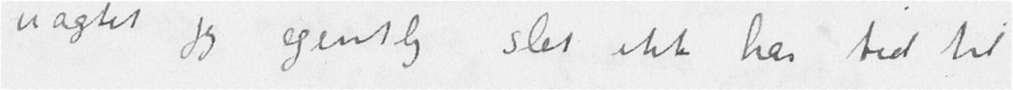uagtet jeg egentlig slet ikke har tid til
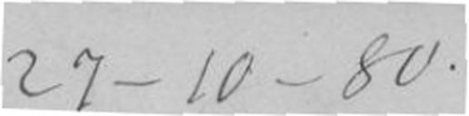27-10-80.
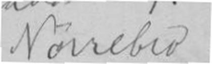Nørrebro.
